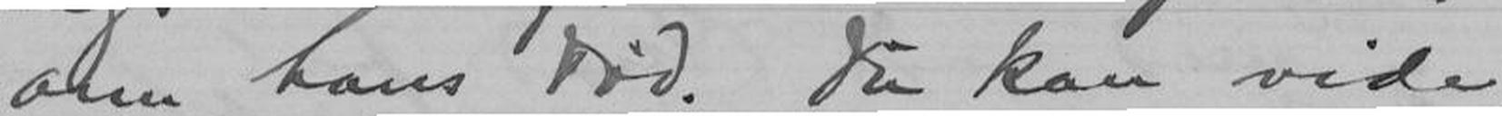om hans Død. Du kan vide,
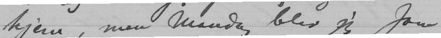hjem, men Mandag blev jeg som
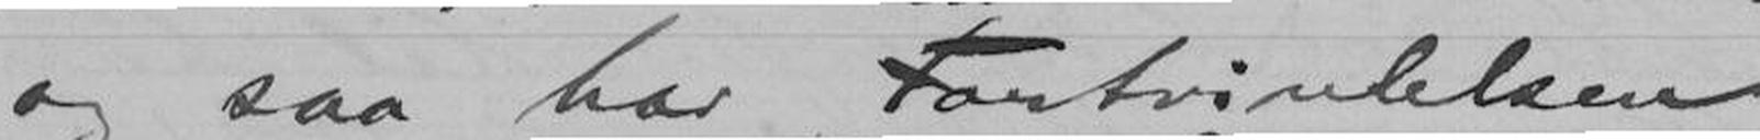og saa har Fortvivlelsen
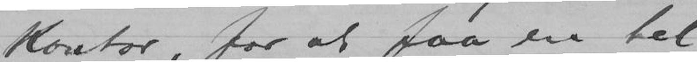Kontor, for at faa en hel
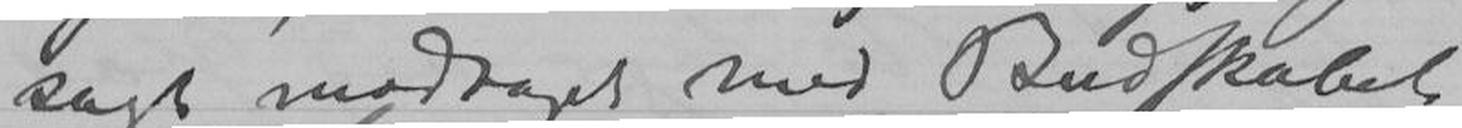sagt modtaget med Budskabet
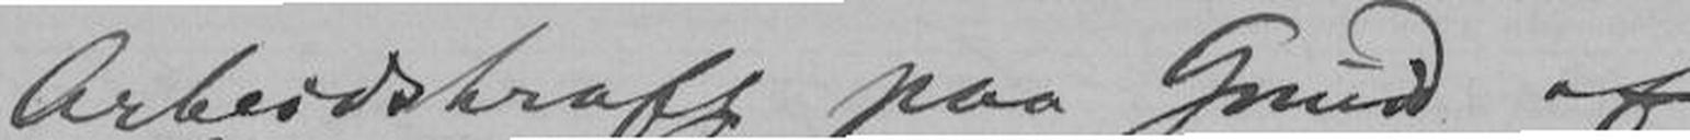Arbeidskraft paa Grund af
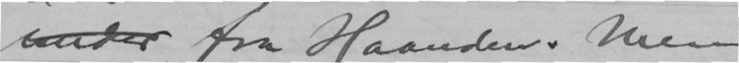under fra Haanden. Men
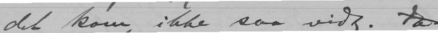det kom ikke saa vidt. Da
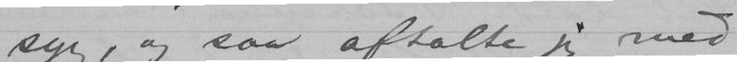syg, og saa aftalte jeg med
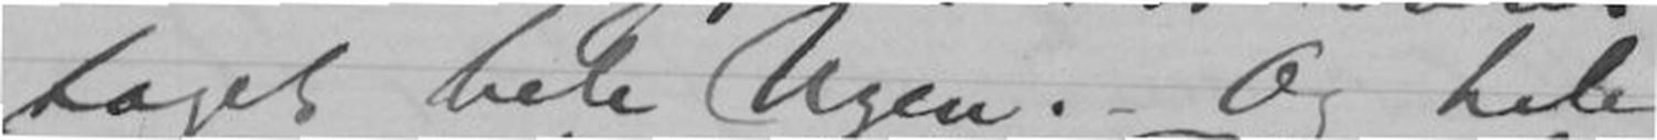taget hele Ugen. Og hele
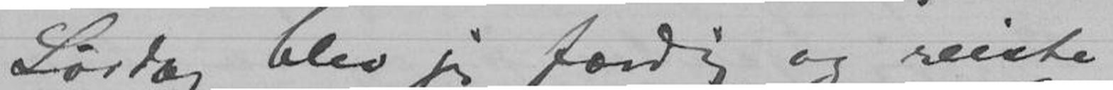Lørdag blev jeg færdig og reiste
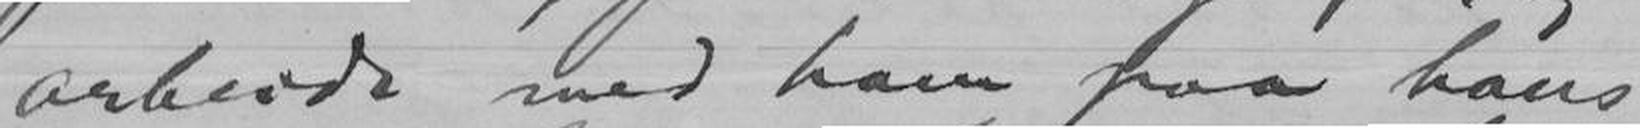arbeide med ham paa hans
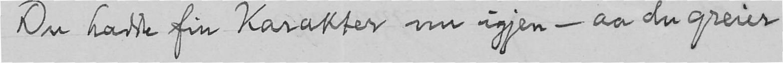Du hadde fin Karakter nu igjen - aa du greier
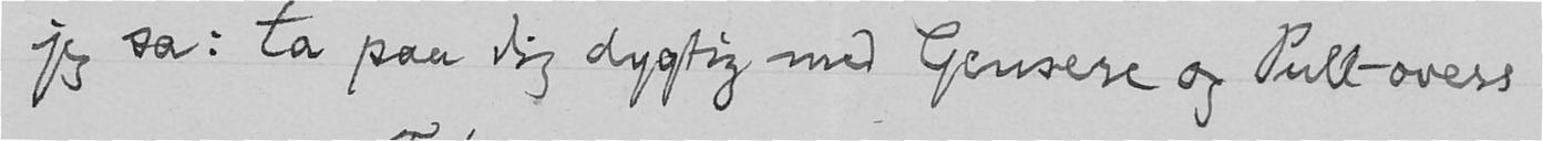jeg sa: ta paa dig dygtig med Gensere og Pull-overs
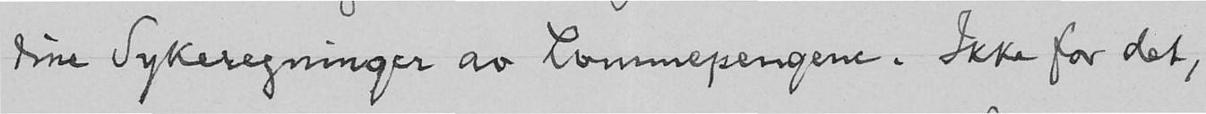dine Sykeregninger av Lommepengene. Ikke for det,
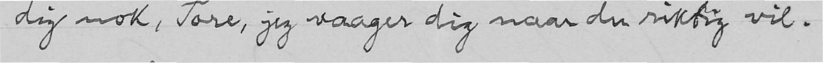dig nok, Tore, jeg vaager dig naar du riktig vil.
